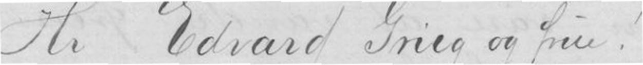Hr Edvard Grieg og frue!
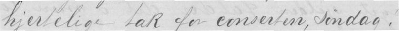hjertelige tak for conserten, søndag!
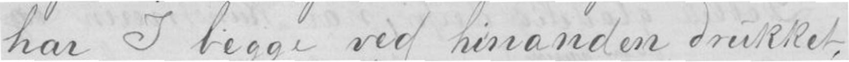har I begge ved hinanden drukket,
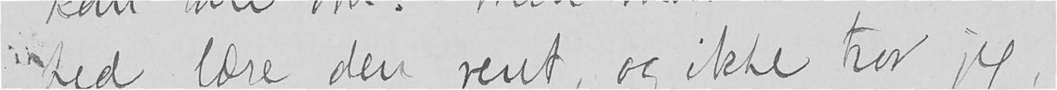hed lære den rent, og ikke tror jeg,
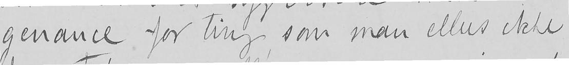genance for ting, som man ellers ikke
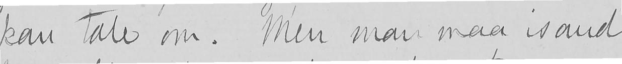kan tale om. Men man maa isand-
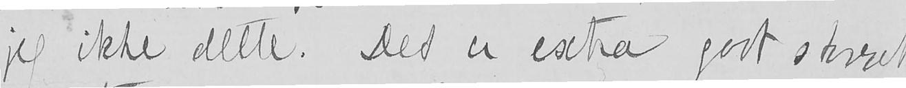jeg ikke dette. Det er extra godt skrevet,
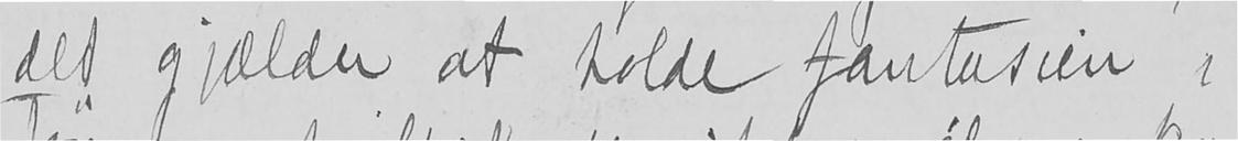det gjælder at holde fantasien i
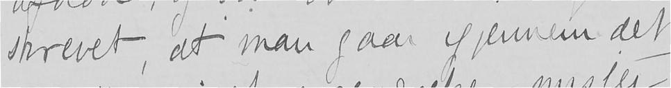skrevet, at man gaar igjennem det
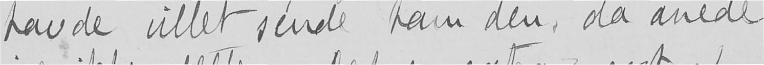havde villet sende ham den, da anede
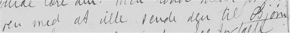ren med at ville sende den til Bjørn-
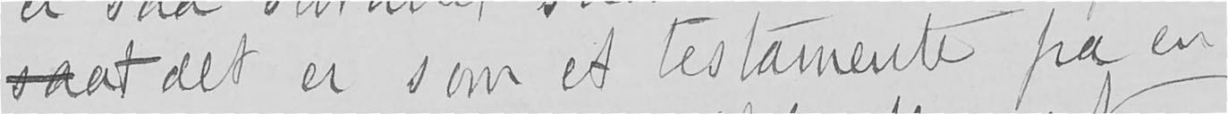saat det er som et testamente fra en
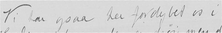Vi har ogsaa her fordybet os i
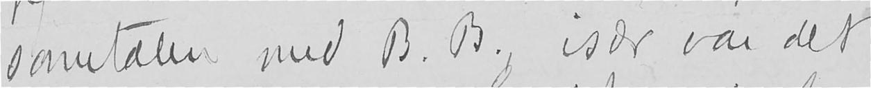samtalen med B. B., især var det
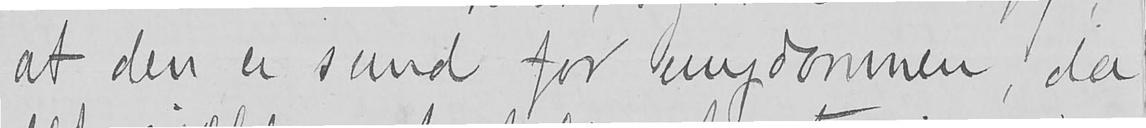at den er sund for ungdommen, da
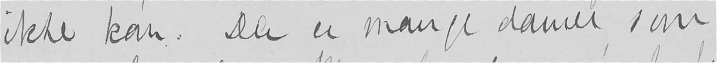ikke kan. Der er mange damer som
